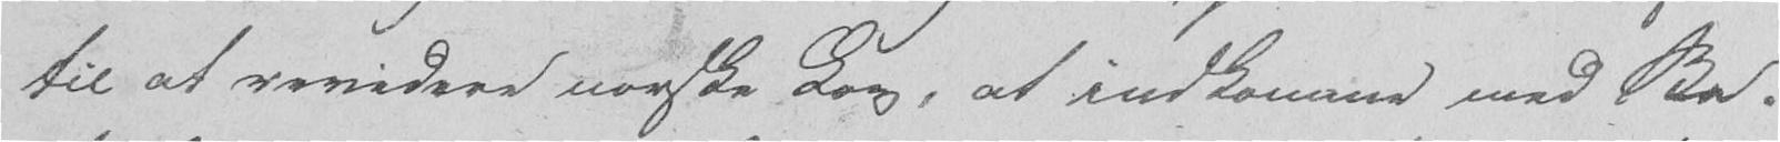til at revidere norske Lov, at indkomme med Be-
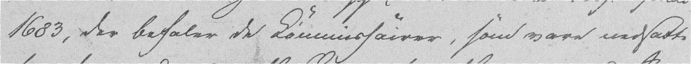1683, der befaler de Kommissairer, som vare nedsatte
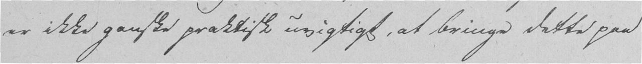er ikke ganske praktisk uvigtigt, at bringe dette paa
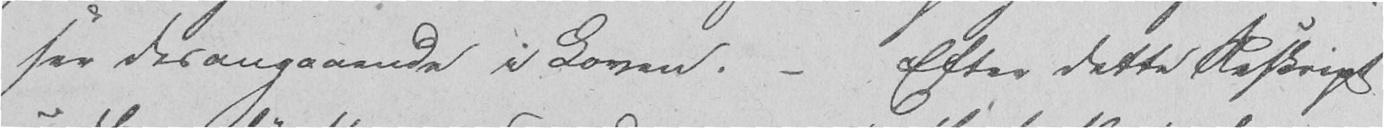ser desangaaende i Loven. - Efter dette Reskript
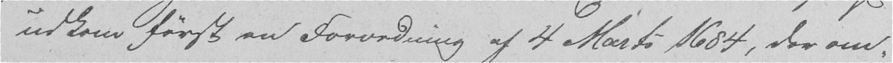udkom først en Forordning af 4 Marti 1684, der om-
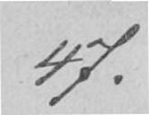47.
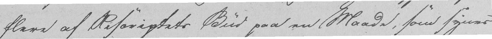flere af Rescriptets Bud paa en Maade, som synes
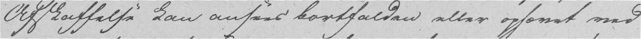Afskaffelse kan ansees bortfalden eller ophævet ved
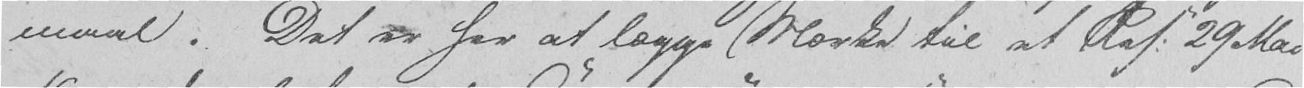maal. Det er her at lægge Mærke til et Res. 29 Mai
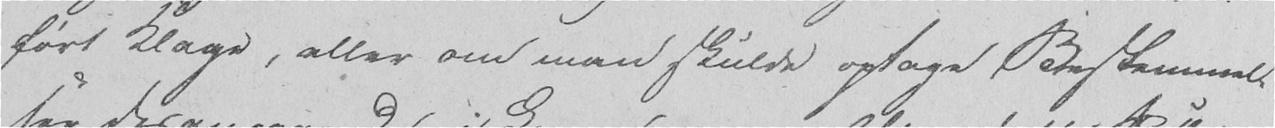ført Klage, eller om man skulde optage Bestemmel-
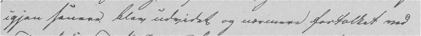igjen senere blev udvidet og nærmere fortolket ved
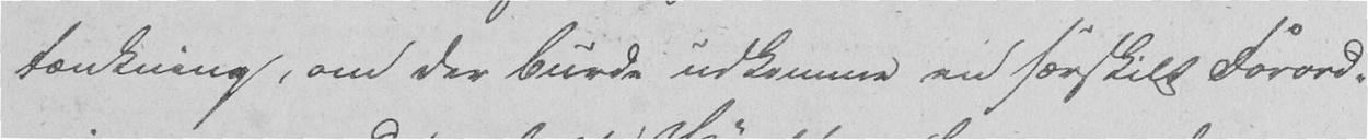tænkning, om der burde udkomme en særskilt Forord-
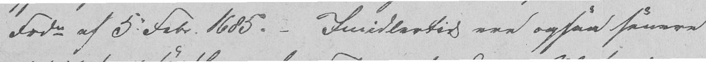Frdn af 5. Febr. 1685. - Imidlertid ere ogsaa senere
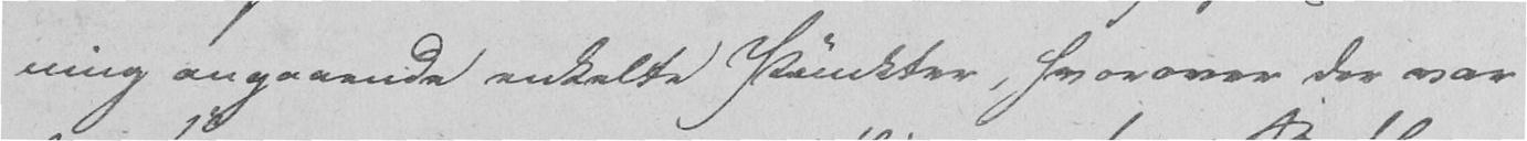ning angaaende enkelte Punkter, hvorover der var
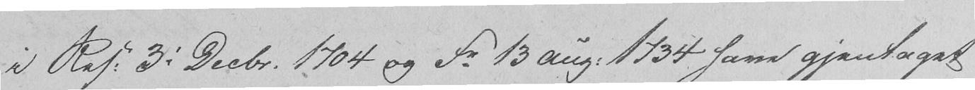i Res. 3. Decbr. 1704 og Fr 13 aug. 1734 have gjentaget
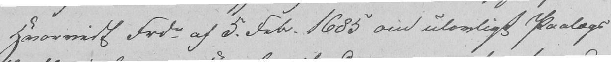Hvorvidt Frdn af 5. Febr. 1685 om ulovligt Paalægs
